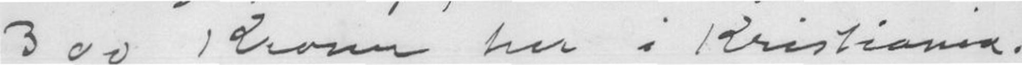300 Kroner her i Kristiania.
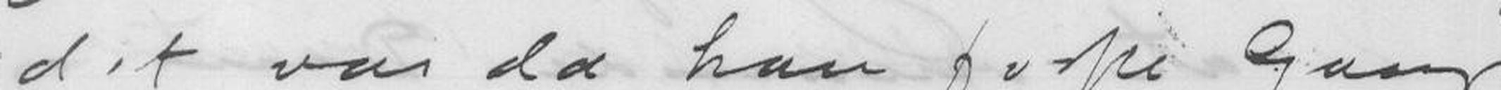det var da han første Gang
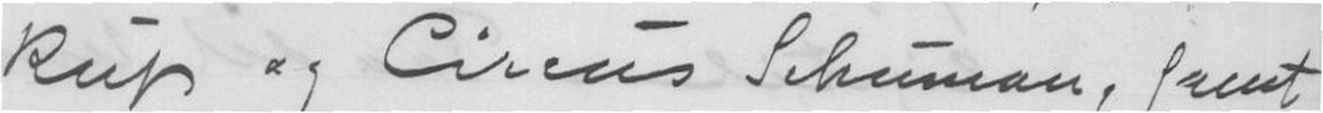kup og Circus Schuman, samt
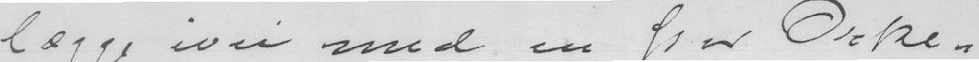lægge ivei med en stor Orke-
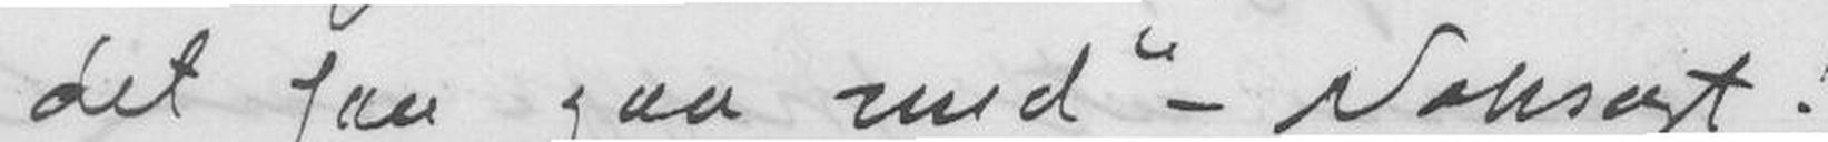det saa gaa med" - Noksagt!-
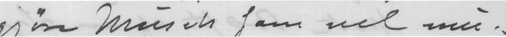gjøre Musik som vel mu-
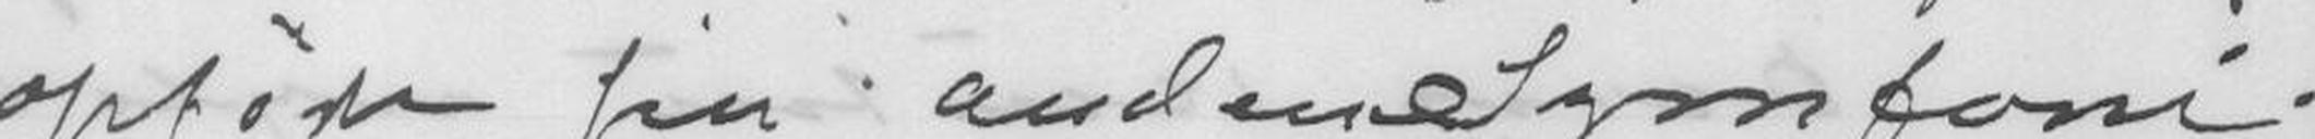opførte sin anden Symfoni.
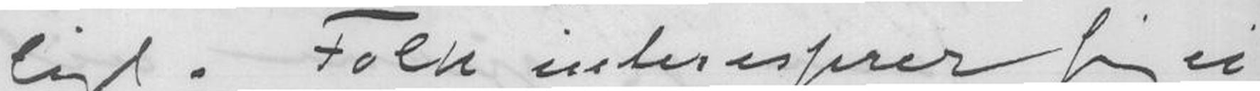ligt. Folk interesserer sig ei
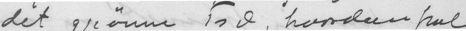det grønnne Træ, hvordan skal
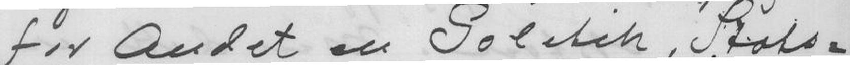for Andet en Politik, Stats-
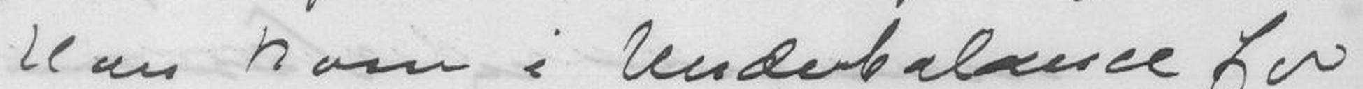Han kom i Underbalance for
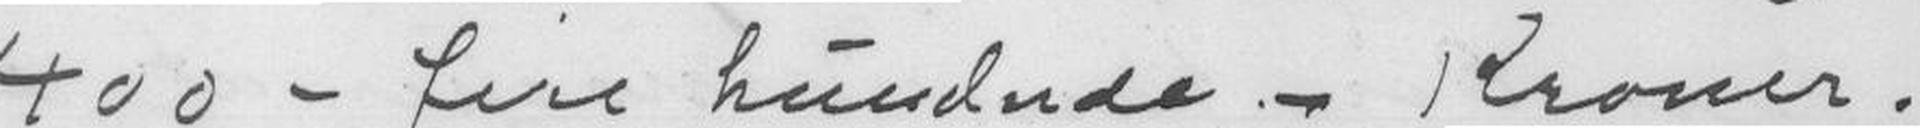400 - fire hundrede - Kroner.
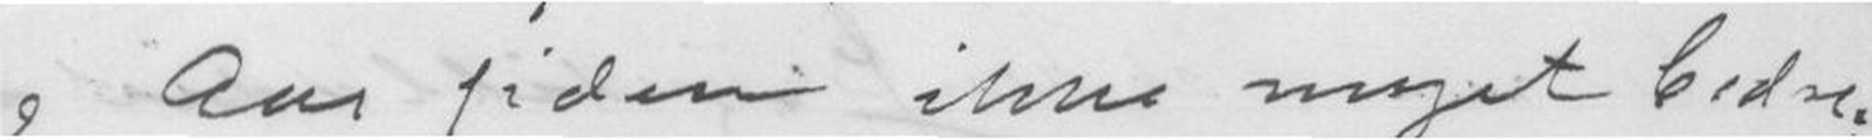ge Aar siden ikke noget bedre;
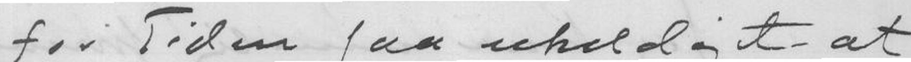for Tiden saa uheldiget at
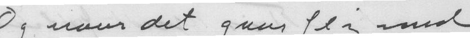"Og naar det gaar slig med
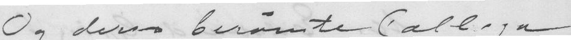Og Deres berømte Collega
